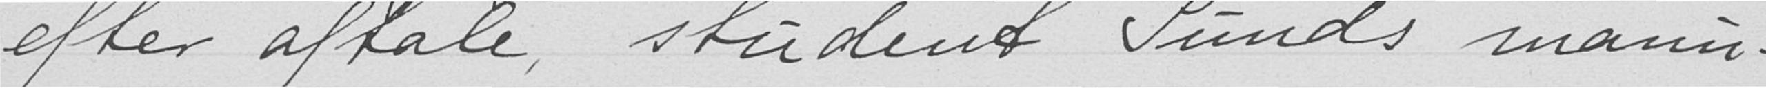efter aftale, student Lunds manu-
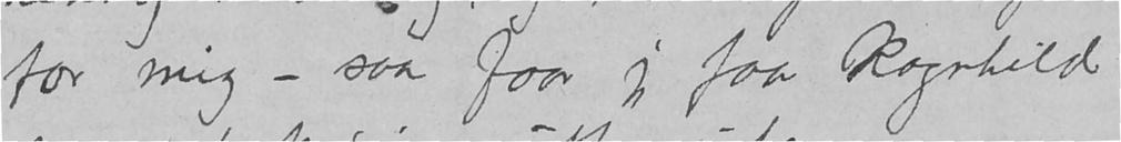for mig – saa faar jeg faa Ragnhild
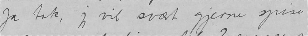Ja tak, jeg vel svært gjerne spise
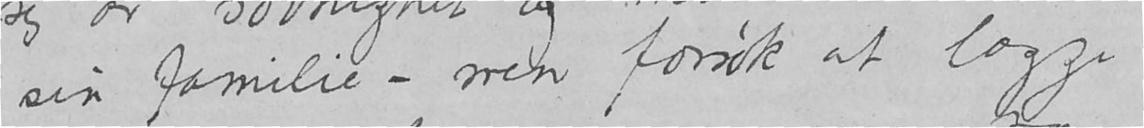sin familie – men forsøk at lægge
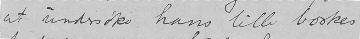at undersøke hans lille boskes
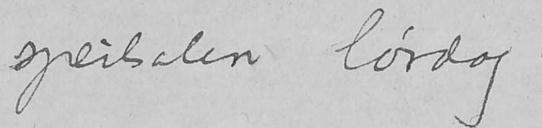speilsalen lørdag.
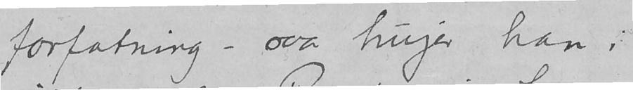forfatning – saa hujer han i
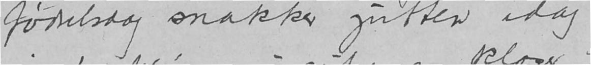fødselsdag snakker gutten idag
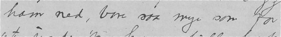ham ned, bare saa mye som for
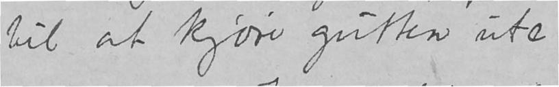til at kjøre gutten ute
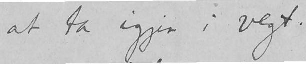at ta igjen i vegt.
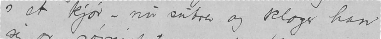i et kjør – no sutrer og klager han
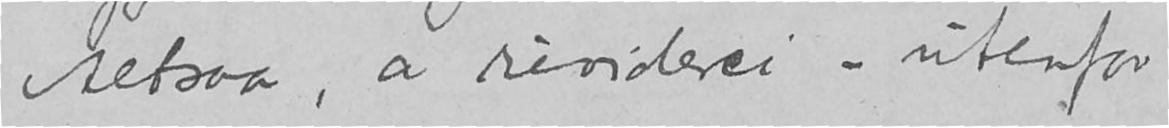Altsaa, av rividerci – utenfor
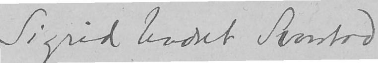Sigrid Undset Svarstad
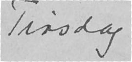Tirsdag
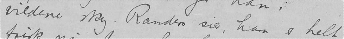vildene sky. Randers sier, han er helt
